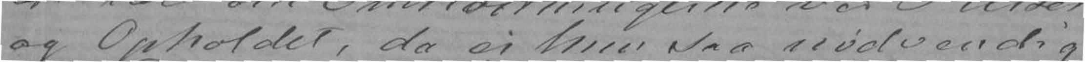og Opholdet, da er hun saa nødvendig
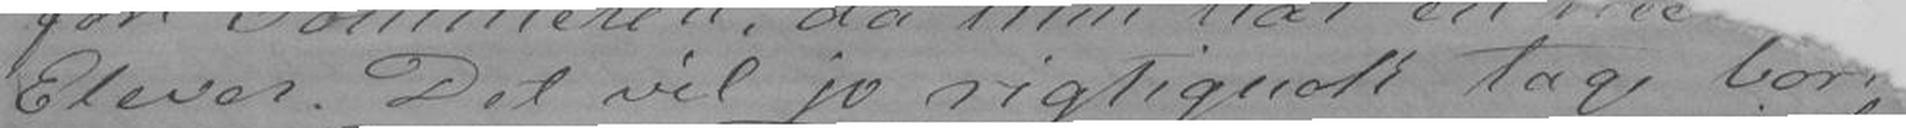Elever, Det vil jo rigtignok tage bor
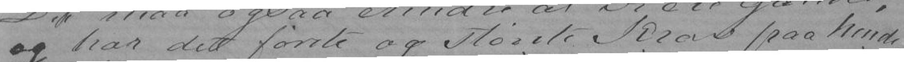og har det første og største Krav paa hende,
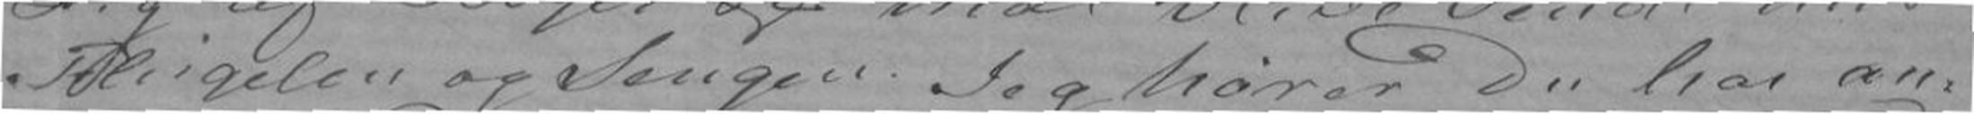Flügelen og Sengen. Jeg hører Du har an-
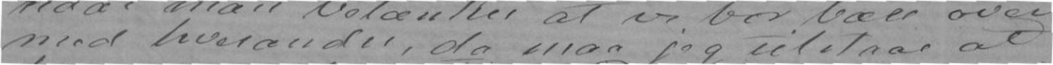med hverandre, da maa jeg tilstaae at
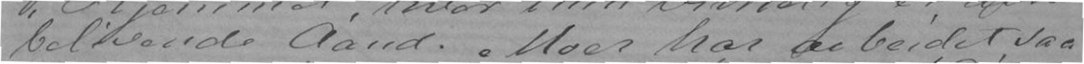belivende Aand. Moer har arbeidet saa
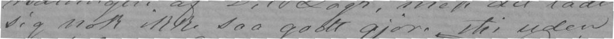sig nok ikke saa godt gjøre, thi uden
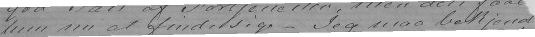hun nu at finde sig. - Jeg maa bekjend
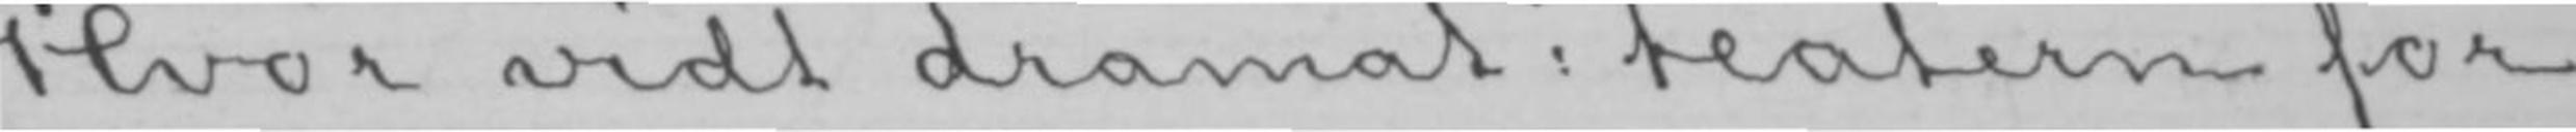Hvor vidt dramat: teatern for
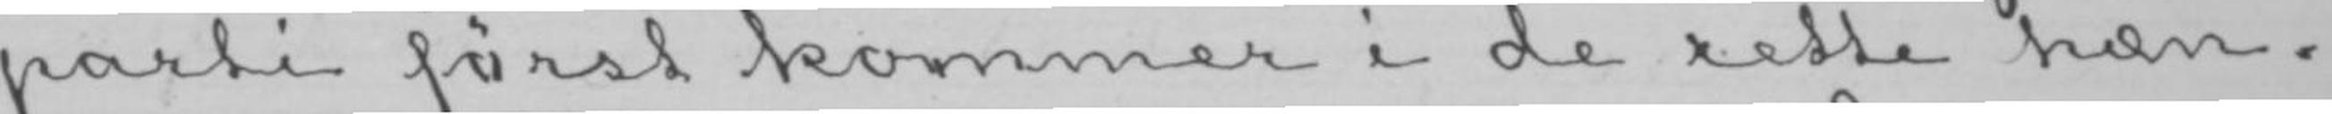parti først kommer i de rette hæn-
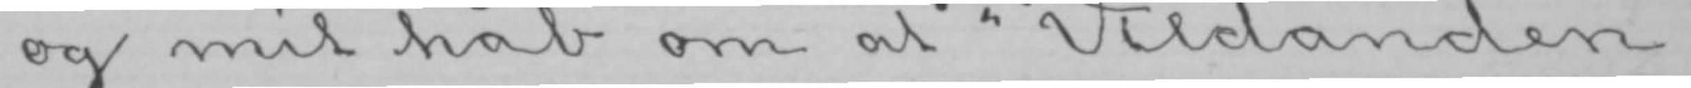og mit håb om at «Vildanden»
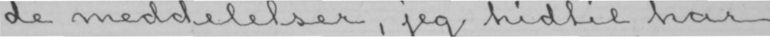de meddelelser, jeg hidtil har
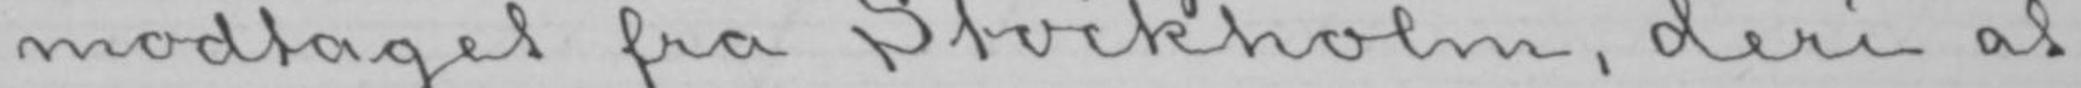modtaget fra Stockholm, deri at
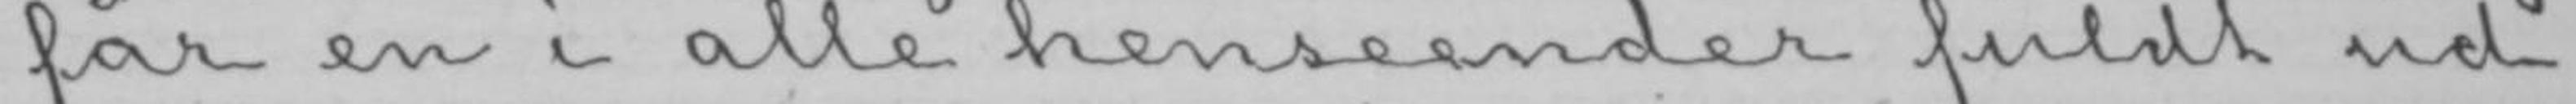får en i alle henseender fuldt ud
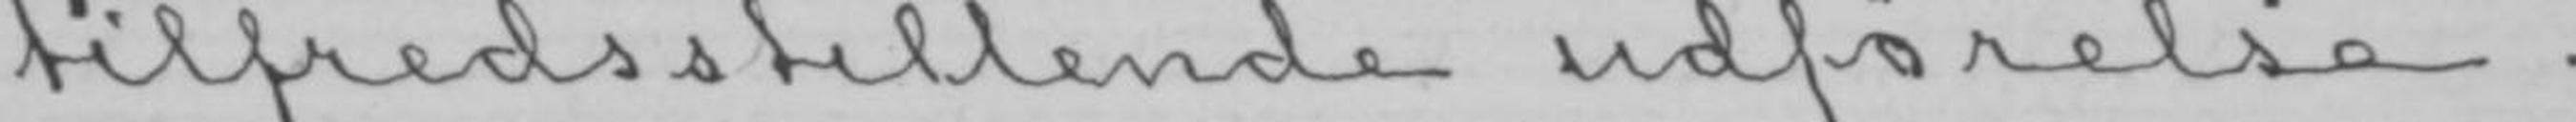tilfredsstillende udførelse.
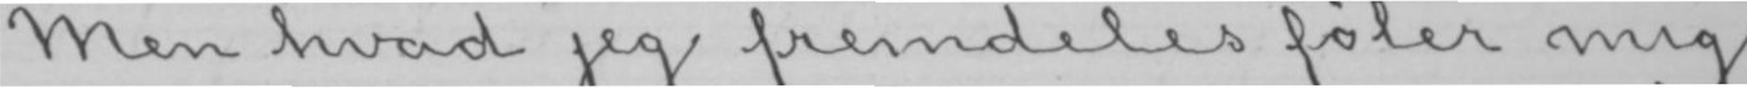Men hvad jeg fremdeles føler mig
